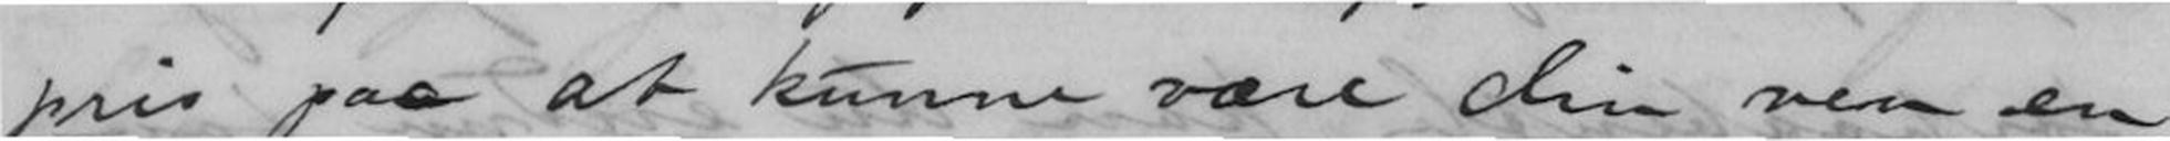pris paa at kunne være din ven, en
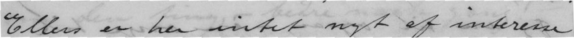Ellers er her intet nyt af interesse,
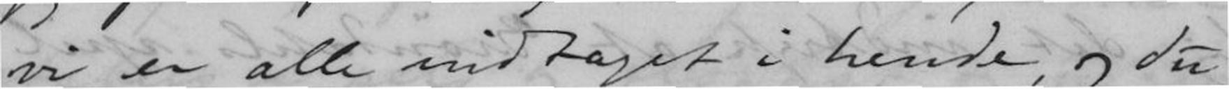vi er alle indtaget i hende, og du
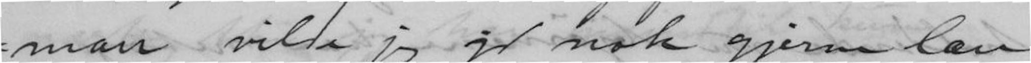marr vilde jeg jo nok gjerne lære
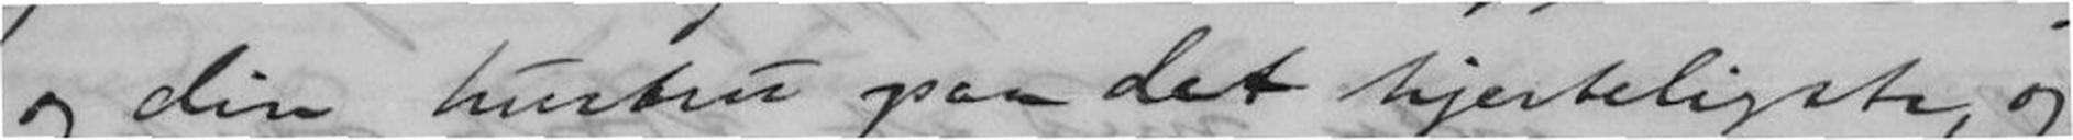og din hustru paa det hjerteligste, og
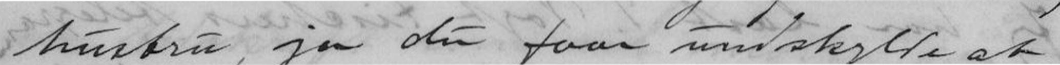hustru, ja du faar undskylde at
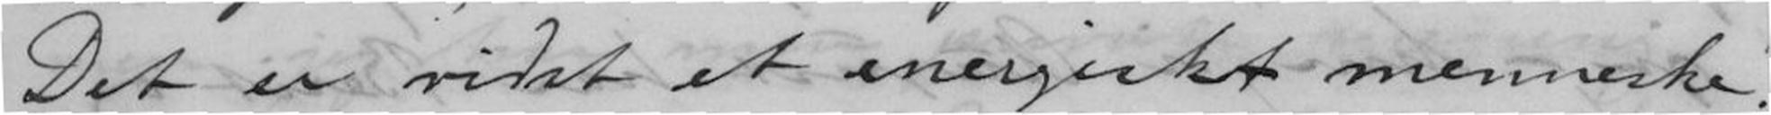Det er vidst et energiskt menneske!
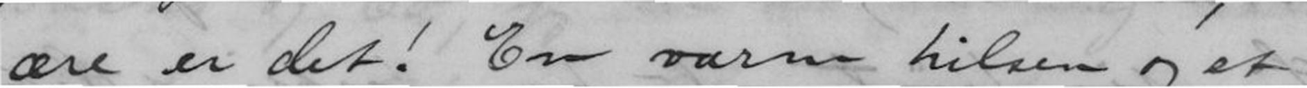ære er det! En varm hilsen og et
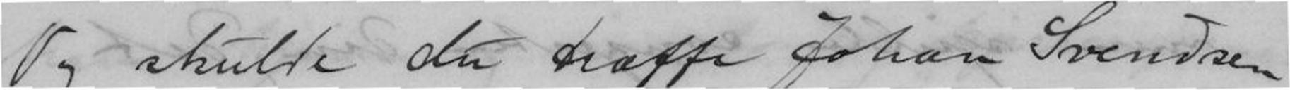Og skulde du træffe Johan Svendsen,
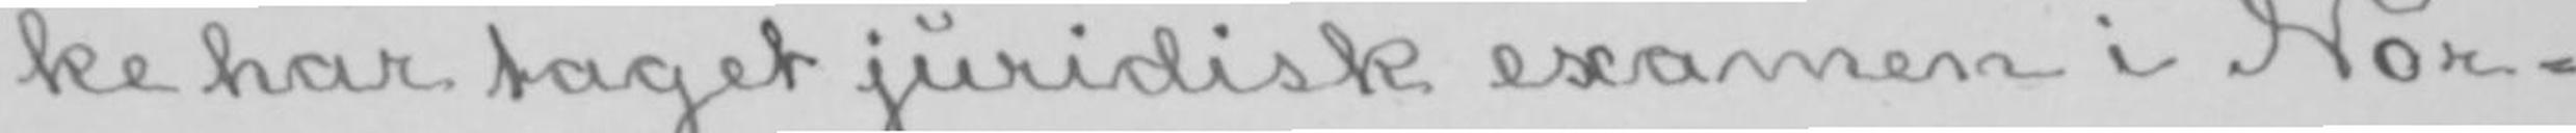ke har taget juridisk examen i Nor-
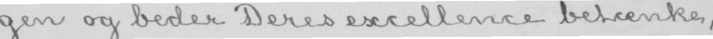gen og beder Deres excellence betænke,
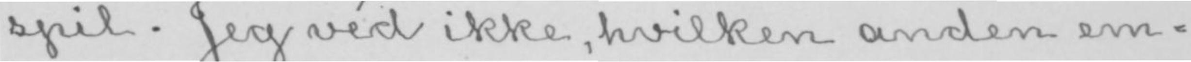spil. Jeg véd ikke, hvilken anden em-
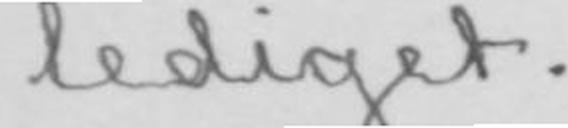lediget.
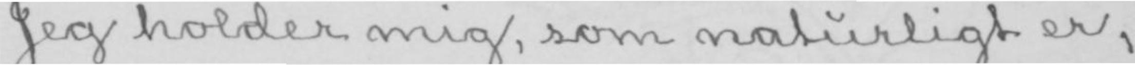Jeg holder mig, som naturligt er,
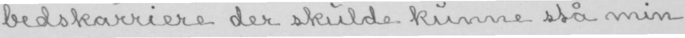bedskarriere der skulde kunne stå min
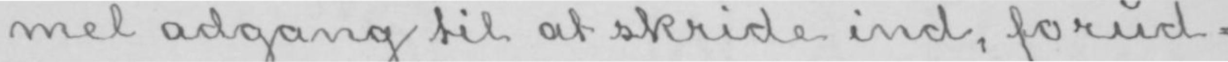mel adgang til at skride ind, forud-
